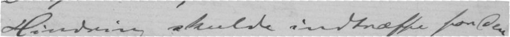Hindring skulde indtræffe før den
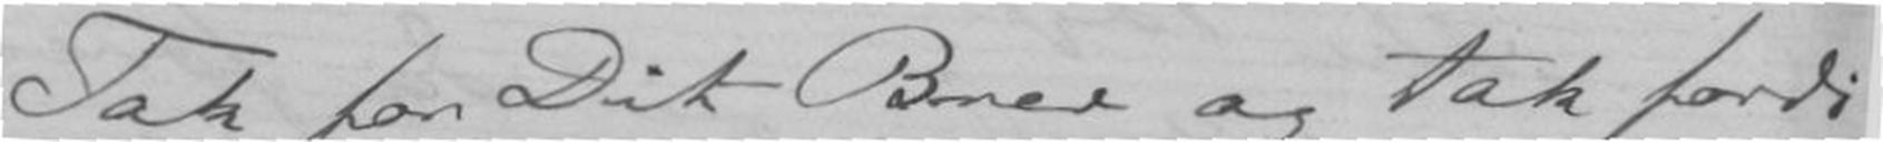Tak for Dit Brev og tak fordi
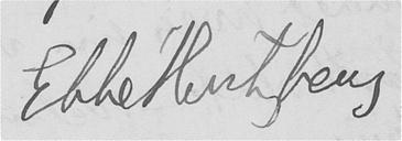Ebbe Hertzberg.
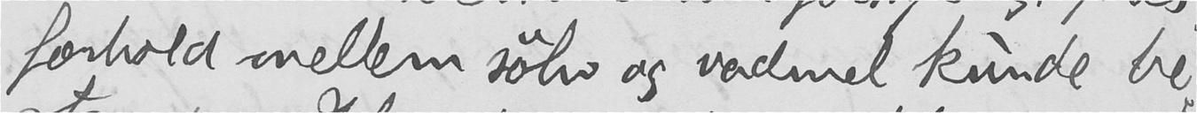forhold mellem sølv og vadmel kunde be-
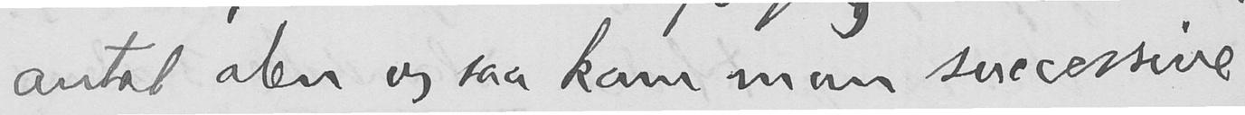antal alen, og saa kom man successive
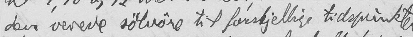den veiede sølvøre til forskjellige tidspunkter.
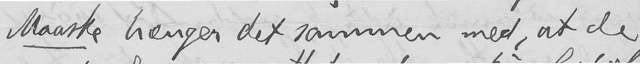Maaske hænger det sammen med, at de
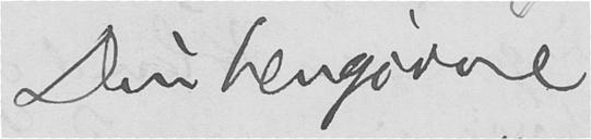Din hengivne
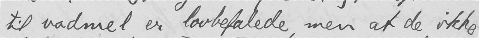til vadmel er lovbefalede, men at de ikke
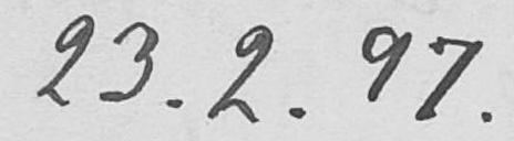23.2.97.
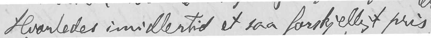Hvorledes imidlertid et saa forskjelligt pris-
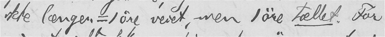ikke længer = 1 øre veiet, men 1 øre tællet. For
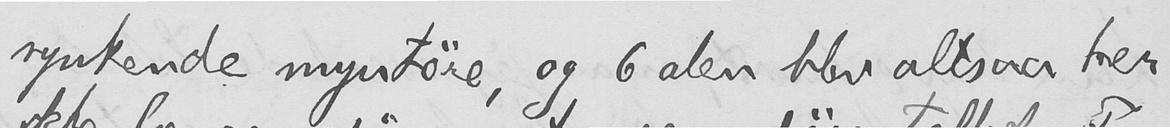synkende myntøre, og 6 alen blev altsaa her
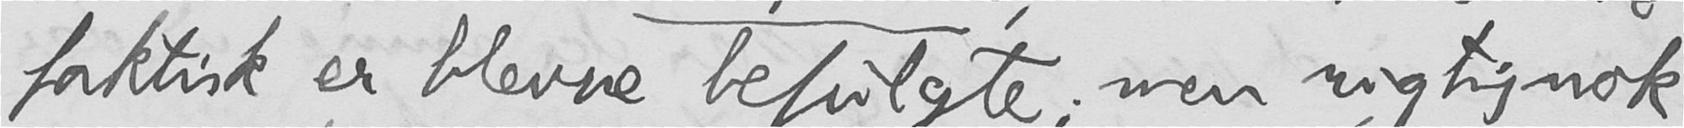faktisk er blevne befulgte; men rigtignok
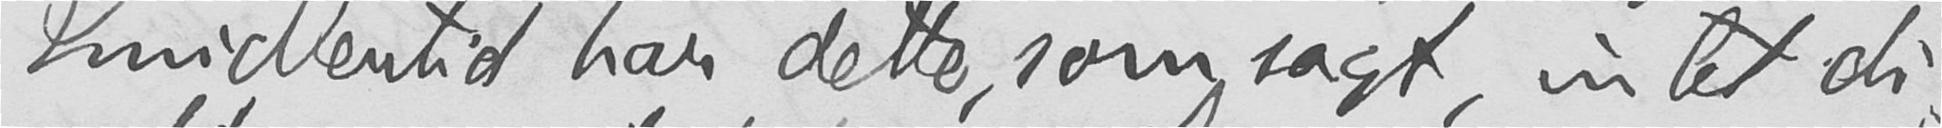Imidlertid har dette, som sagt, intet di-
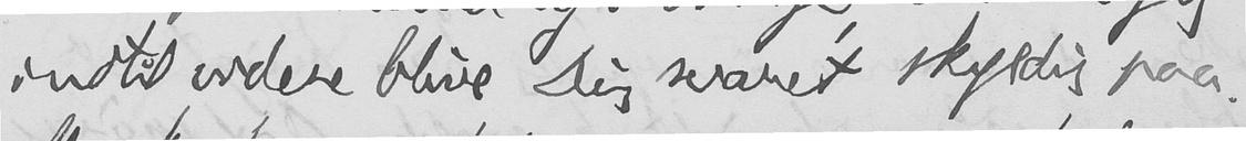indtil videre blive Dig svaret skyldig paa.
